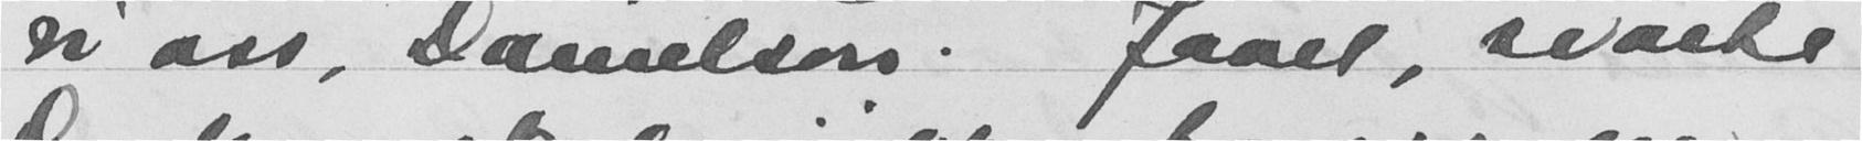vi oss, Danielsen. Javel, svarte
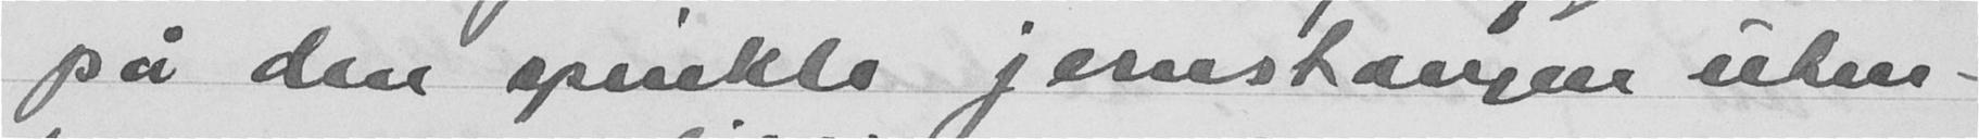på den spinkle jesushaugen uten-
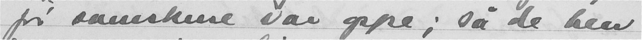før svenskene var oppe; så de blev
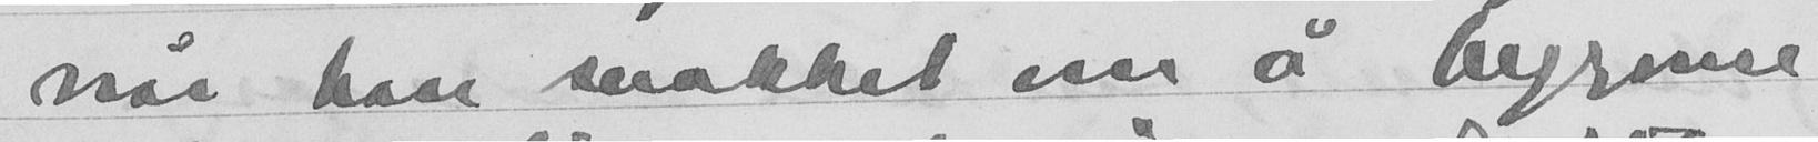når han snakket om å begynne
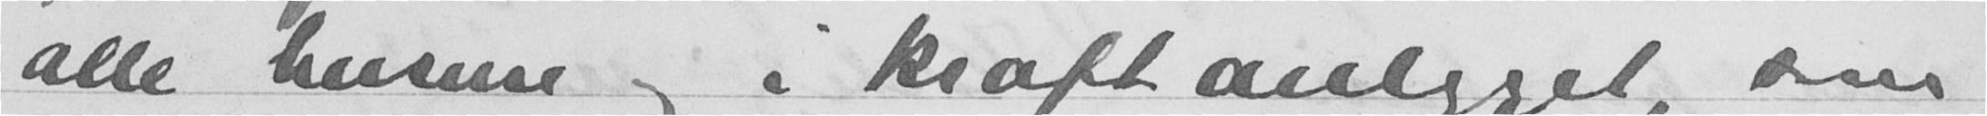alle husene og i kraftanlegget, som
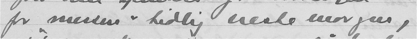for "messen" tidlig neste morgen,
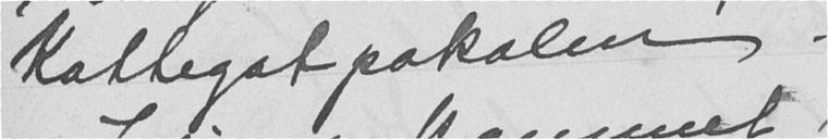Kattegatpokalen.
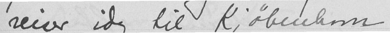reiser idag til Kjøbenhavn
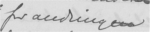forandringen
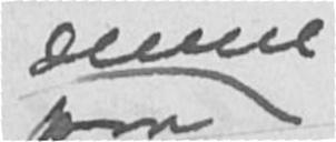denne
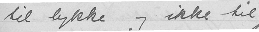til lykke og ikke til
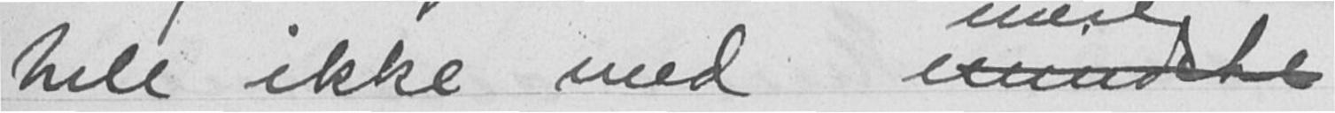hele ikke med mindste
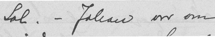Sol. - Johan var om
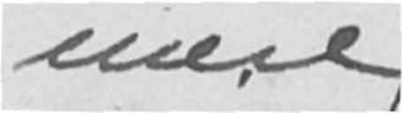mere
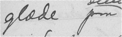glæde paa
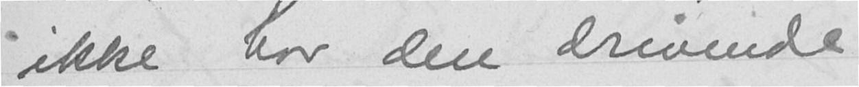ikke har den drivende
